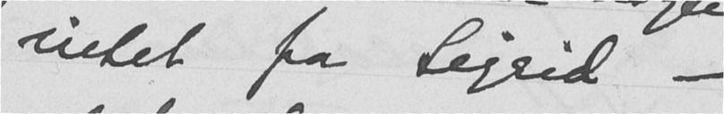intet fra Sigrid -
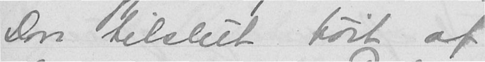Don tilslut høit af
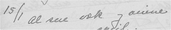15/1 Al sne væk og veiene
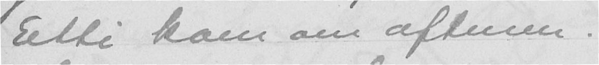Etti kom om aftenen.
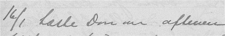16/1 Læste Don om aftenen
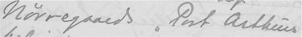Nørregaards "Port Arthurs
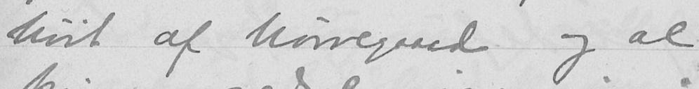høit af Nørregaard og al
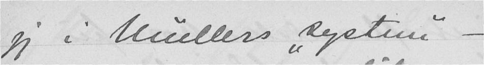jeg i Müllers "system" -
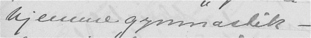hjemmegymnastik -
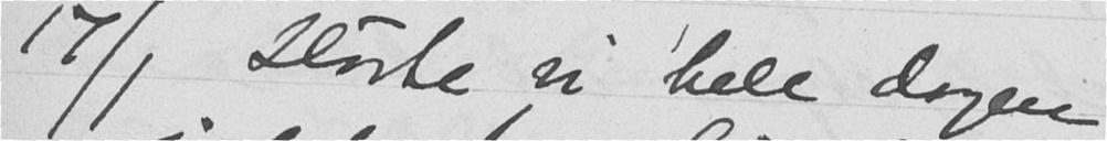17/1 Hørte vi hele dagen
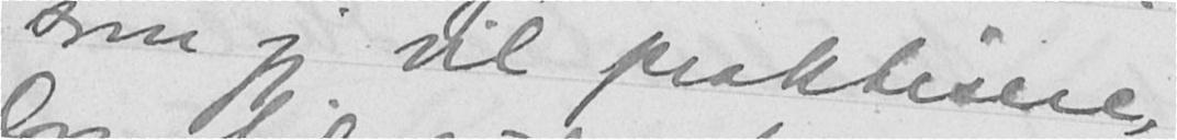som jeg vil praktisere,
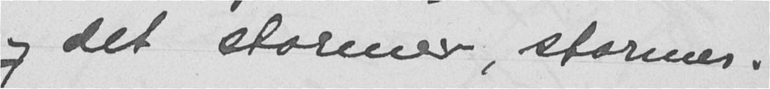og det stormer, stormer.
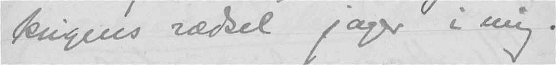krigens rædsel jager i mig.
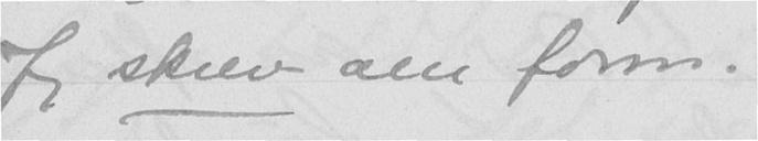Jeg skrev om form.
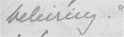beleiring."
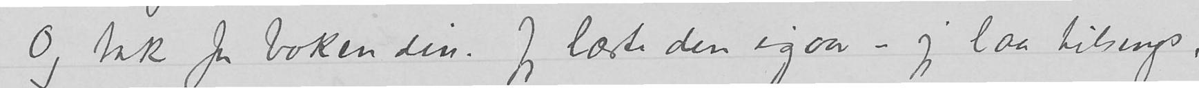Og tak for boken din. Jeg læste den igaar – jeg laa til sengs,
